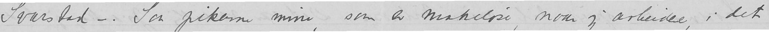Svarstad - Saa pikerne mine, som er makeløse, naar jeg arbeider, i det
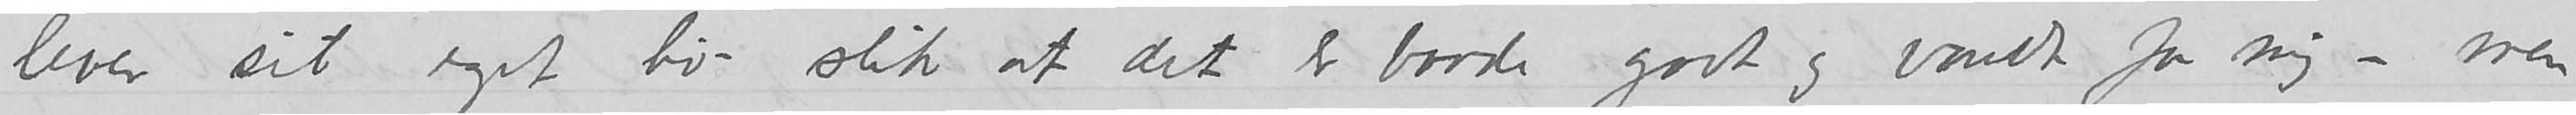lever sit eget liv slik at det er baade godt og vondt for mig - men
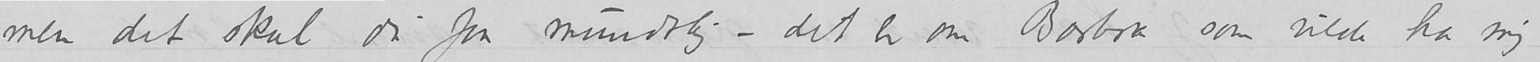men det skal du faa mundtlig - det er om Barbra som vilde ha mig
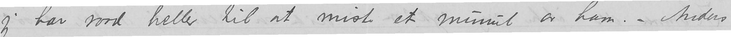jeg har raad  heller til at miste et minut av ham. – Anders
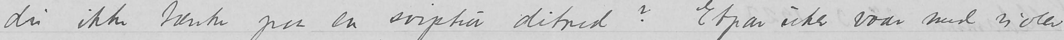du ikke tænke paa en sviptur ditned? Etpar uker vaar med violer
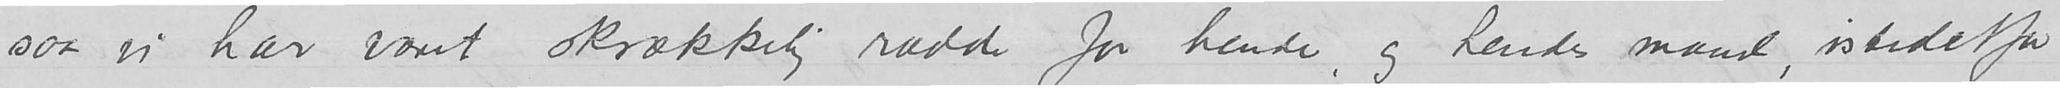saa vi har været skrækkelig rædde for hende, og hendes mand, istedetfor
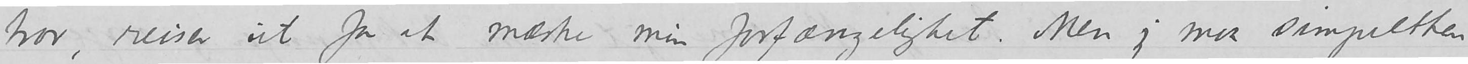tror, reiser ut for at mærke min forfængelighet. Men jeg maa simpelthen
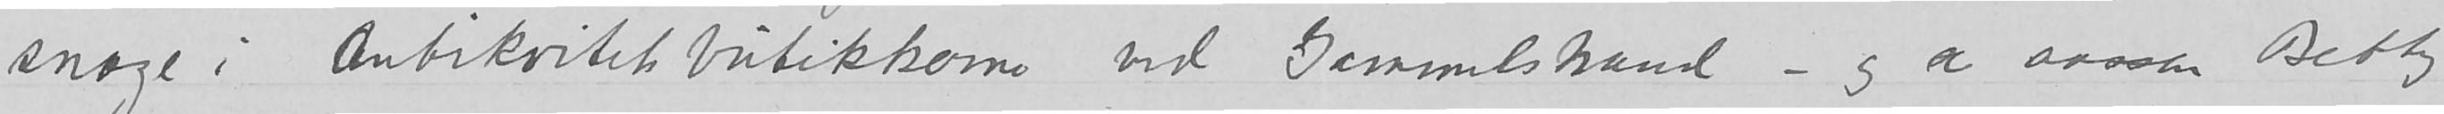snoge i antikvitetsbutikkerne ved Gammelstrand – og se aassen Betty
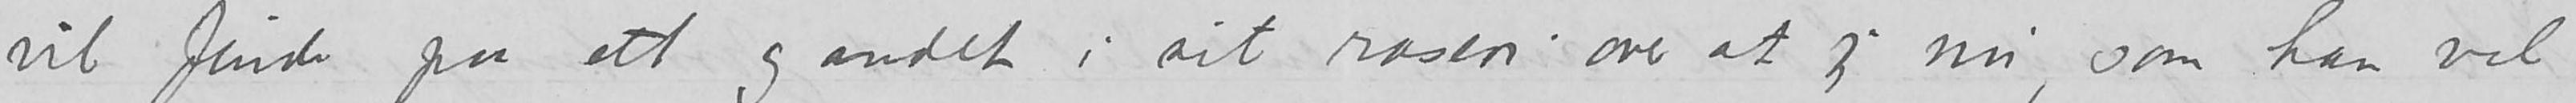vil finde paa ett og andet i sit raseri over at jeg nu, som han vel
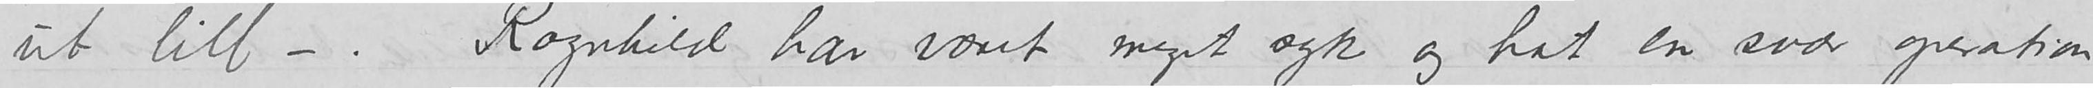ut litt - Ragnhild har været meget syk og hat en svær operation,
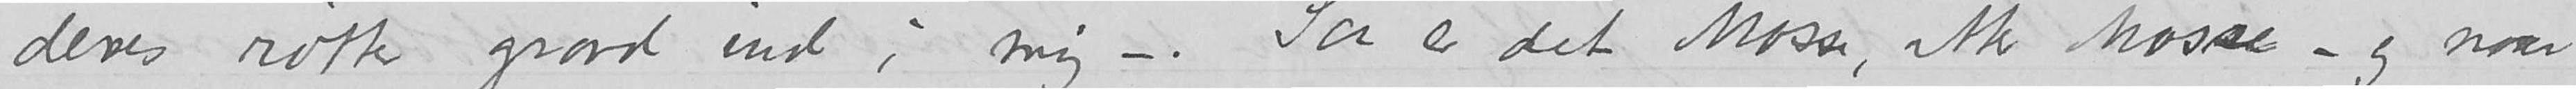deres røtter gravd ind i mig -. Saa er det Mosse, atter Mosse - og naar
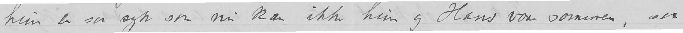hun er saa syk som nu kan ikke hun og Hans være sammen, saa
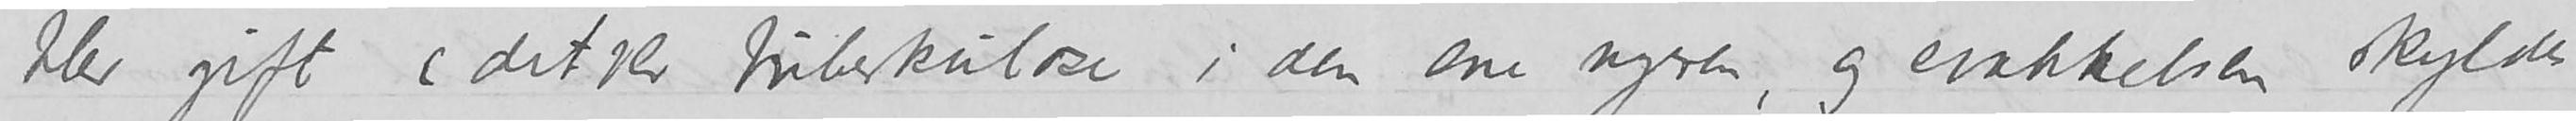blev gift (det var tuberkulose i den ene nyren, og svækkelsen skyldes
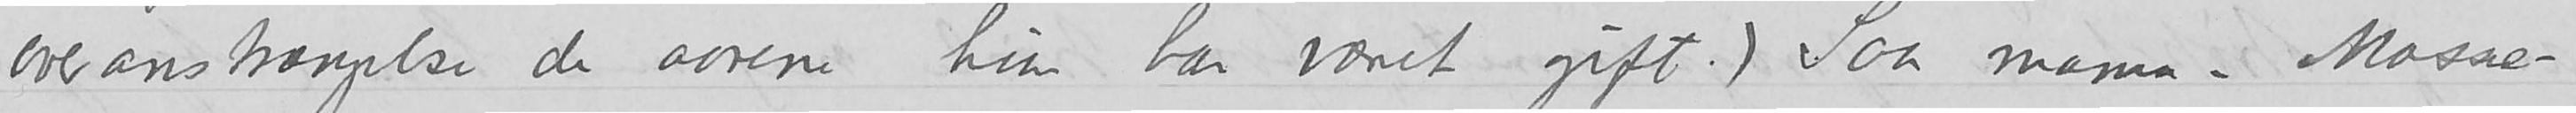overanstrængelse de aarene hun har været gift.) Saa mama - Mosse-
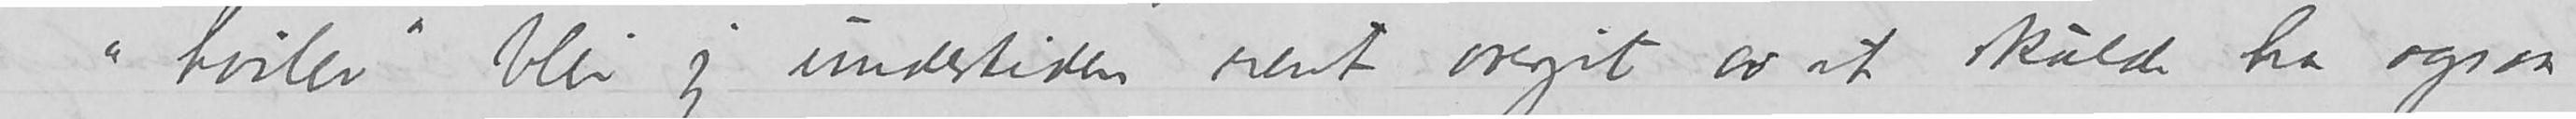jeg «hviler» blir jeg undertiden rent overgit av at skulde ha ogsaa
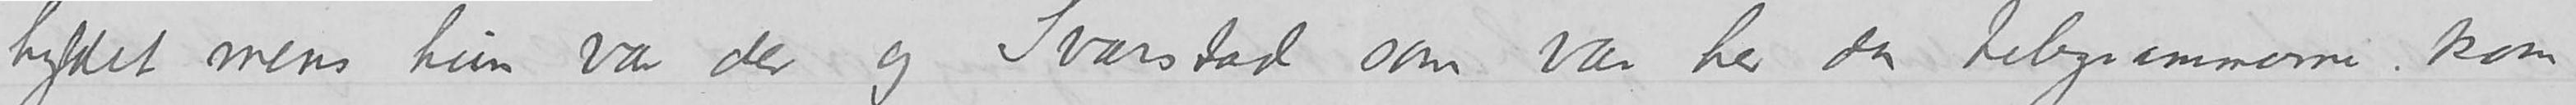hyldet mens hun var der og Svarstad som var her da telegrammerne kom
